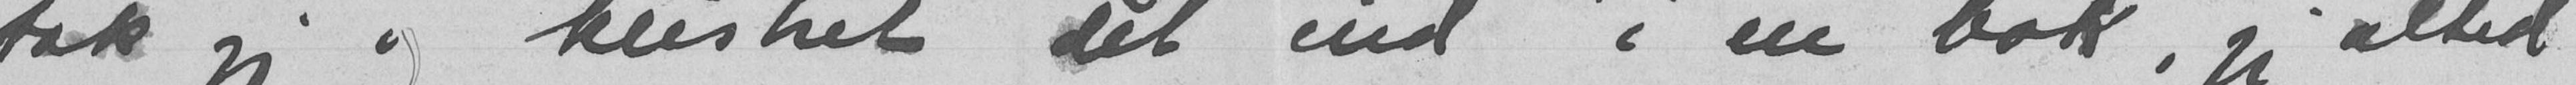tok jeg og klistret det ind i en bok, jeg altid
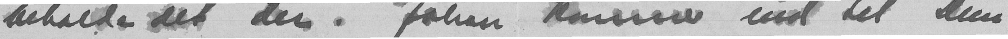beholde det der. Johan kommer ind til Dem
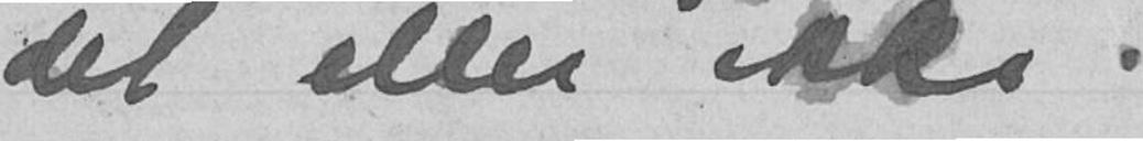det eller ikke.
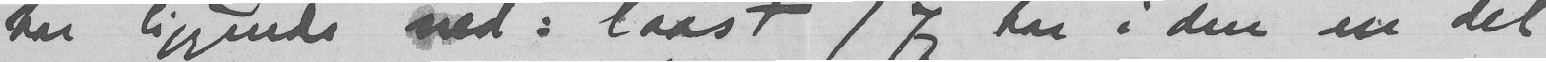har liggende ned-laast (Jeg har i den en del
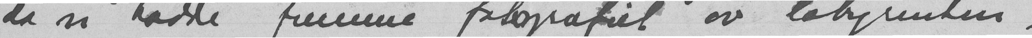da vi hadde fremme fotografiet av labyrinten,
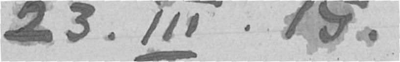23. III. 15.
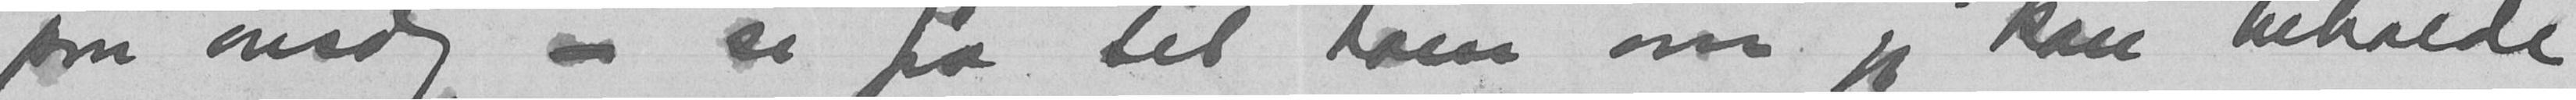paa onsdag - si fra til ham om jeg kan beholde
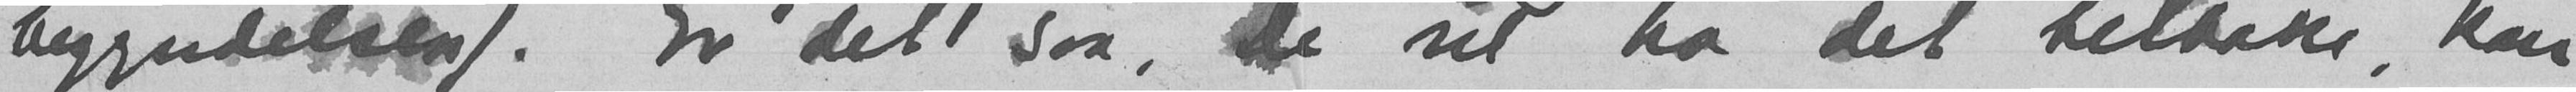begyndelsen). Er det saa, De vil ha det tilbake, kan
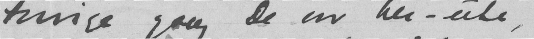Forrige gang De var her-ute,
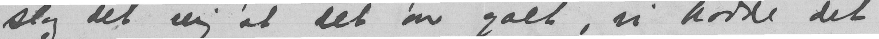slog det mig at det var galt, vi hadde det
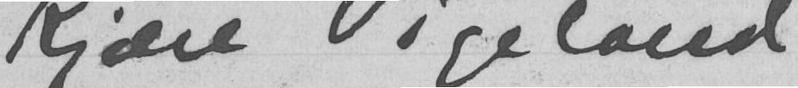Kjære Vigeland
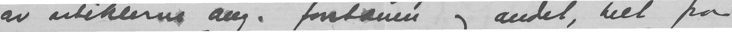av artiklene ang. fontænen og andet, helt fra
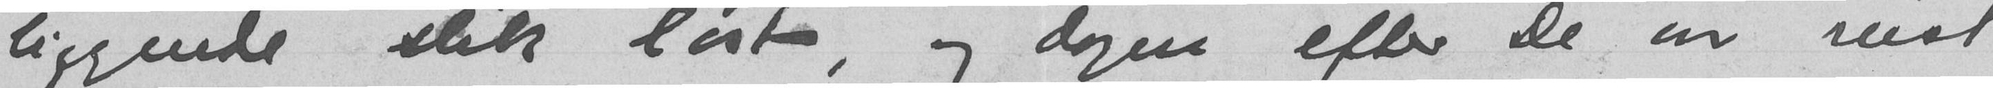liggende slik løst, og dagen efter De var reist
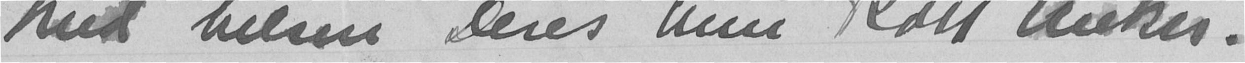Med hilsen Deres Nini Roll Anker.
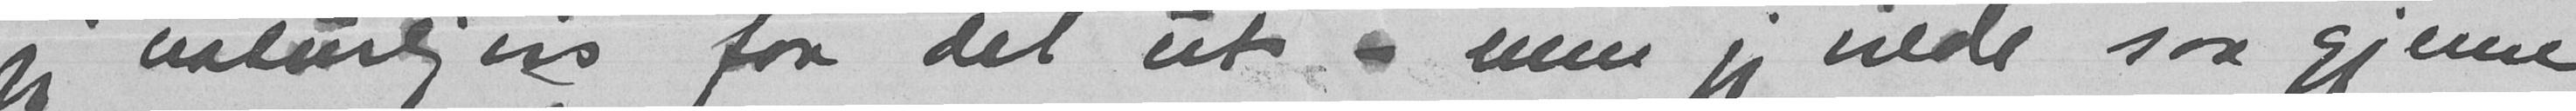jeg naturligvis faa det ut - men jeg vilde saa gjerne
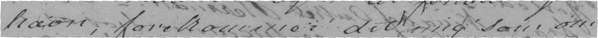havn; forekommer det mig som om
